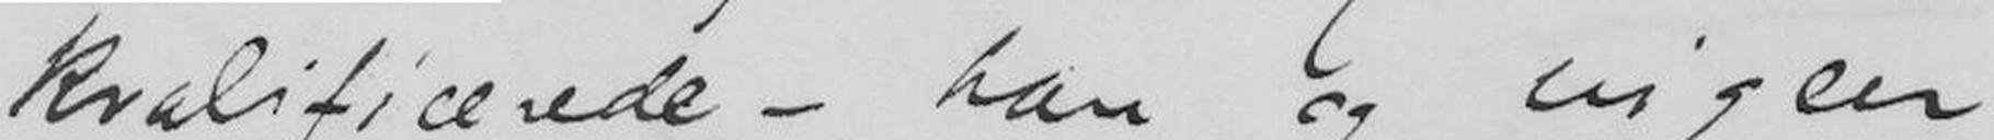kvalificerede - han og ingen
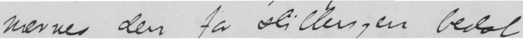nevnes den for stillingen bedst
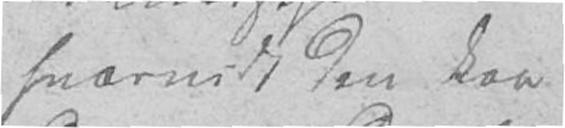hvorvidt den kan
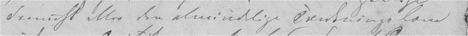Fornuft eller den almindelige Tænknings Love
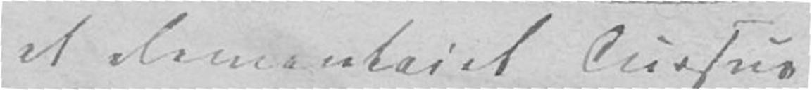et demonstrativt Cursus
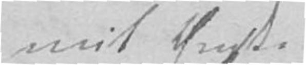mit Ønske
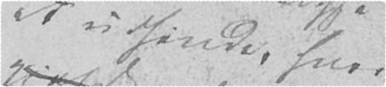at udfinde, hvor
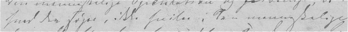hvad der søges, ikke hviler i den menneskelige
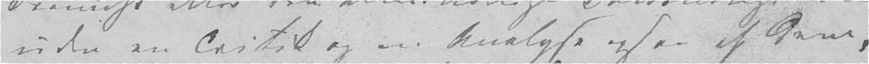uden en Critik og en Analyse ogsaa af dem.
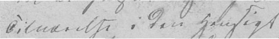Tilværelse i den Hensigt
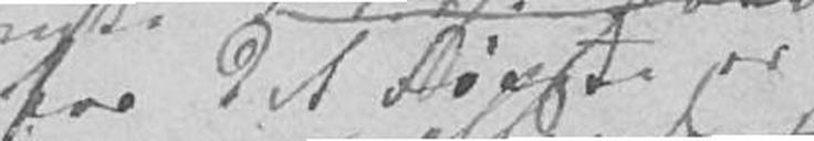for det Første er
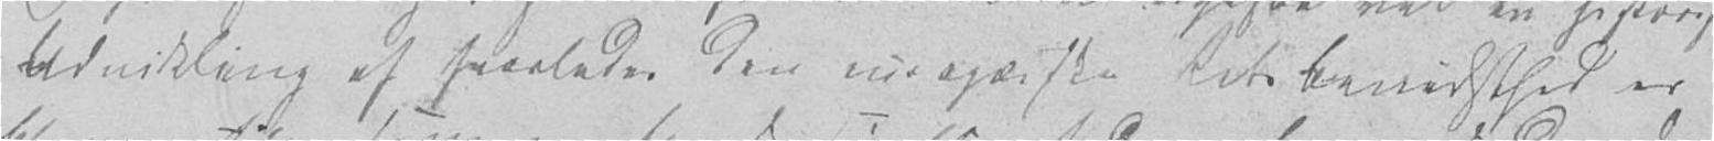Udvikling af hvorledes den inkjærske Retsbevidsthed er
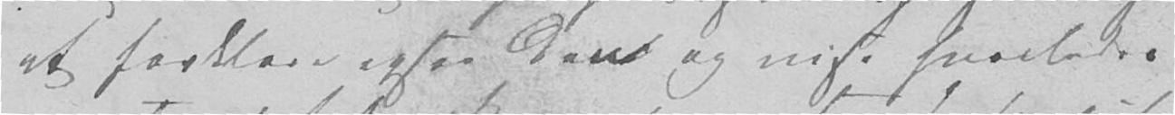at forklare ogsaa den og vise hvorledes
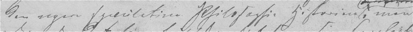den nyere speculative Philosophie Historien, men
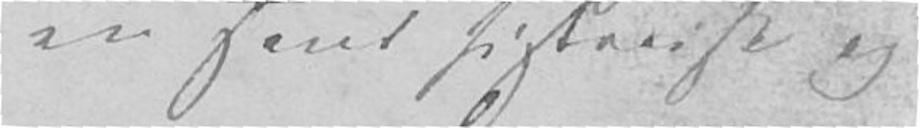en sand historisk og
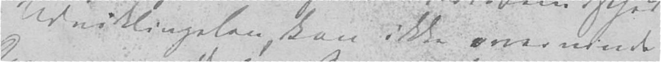Udviklingsplan, kan ikke overvinde
